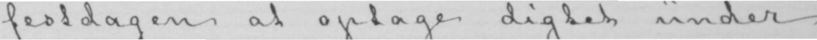festdagen at optage digtet under
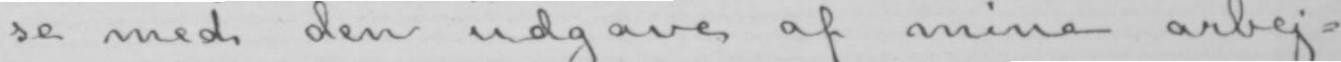se med den udgave af mine arbej-
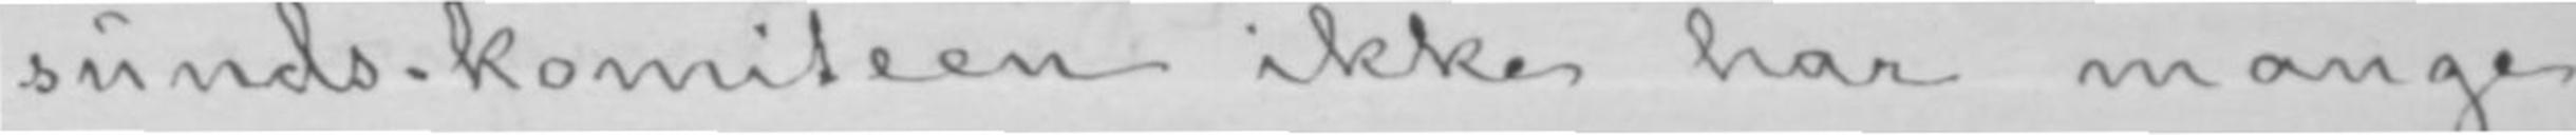sunds-komiteen ikke har mange
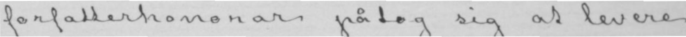forfatterhonorar påtog sig at levere
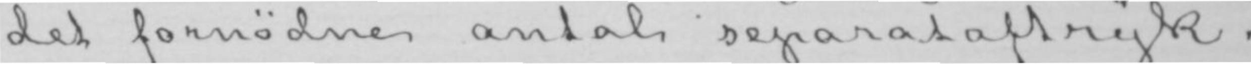det fornødne antal separataftryk.
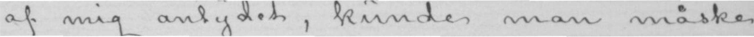af mig antydet, kunde man måske
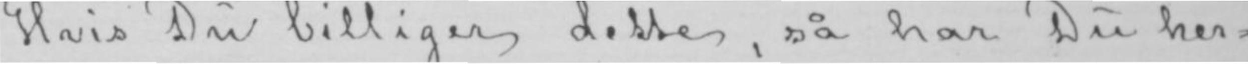Hvis Du billiger dette, så har Du her-
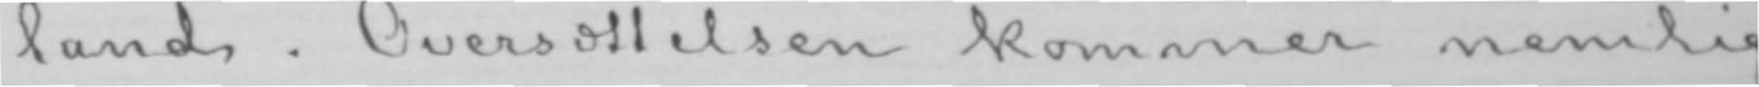land. Oversættelsen kommer nemlig
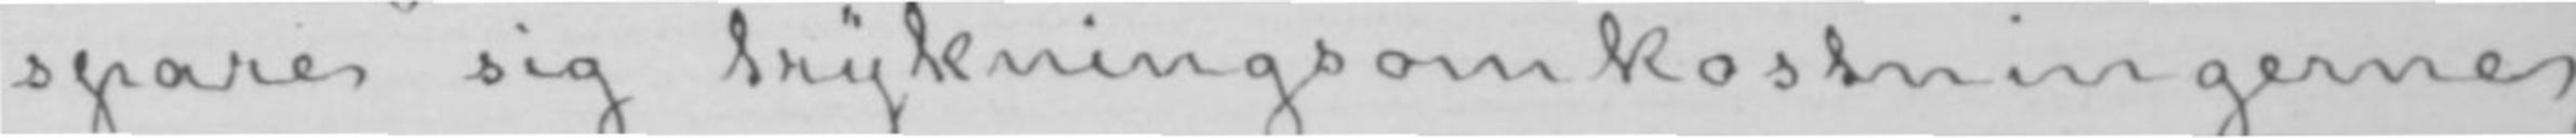spare sig trykningsomkostningerne,
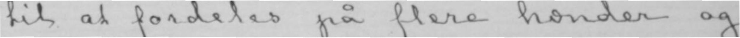til at fordeles på flere hænder og
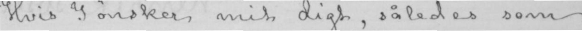Hvis I ønsker mit digt, således som
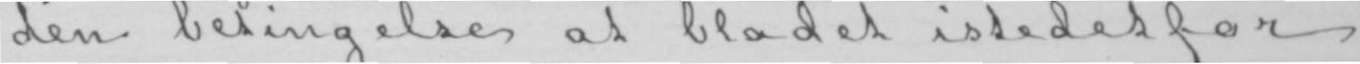den betingelse at bladet istedetfor
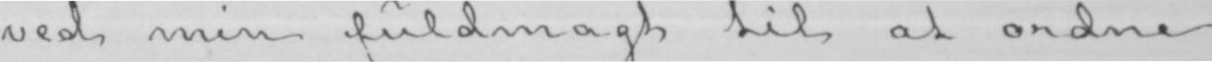ved min fuldmagt til at ordne
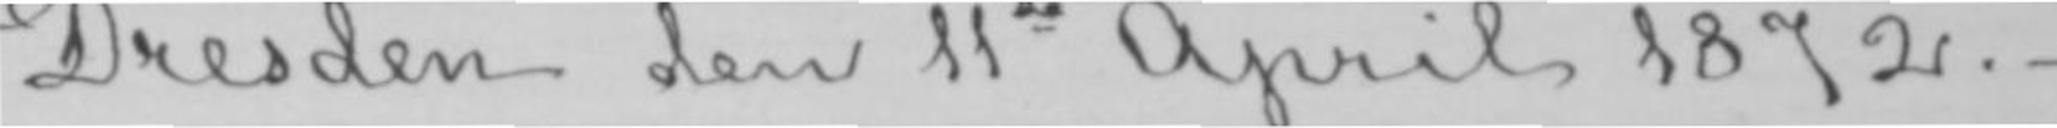Dresden den 11te April 1872.-
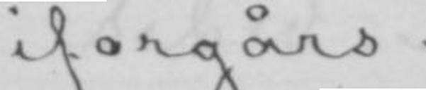iforgårs.
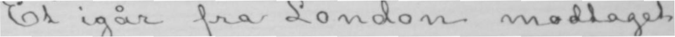Et igår fra London modtaget
